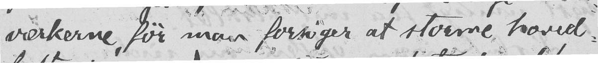værkerne, før man forsøger at storme hoved-
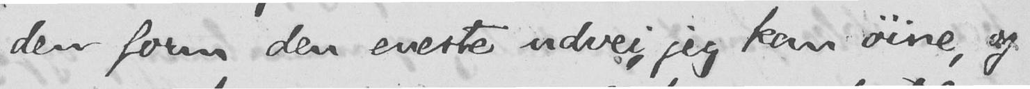den form den eneste udvei, jeg kan øine, og
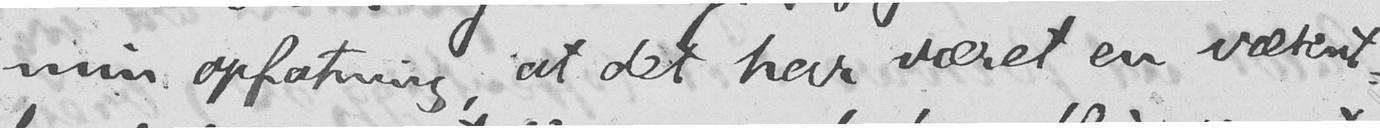min opfatning, at det har været en væsent-
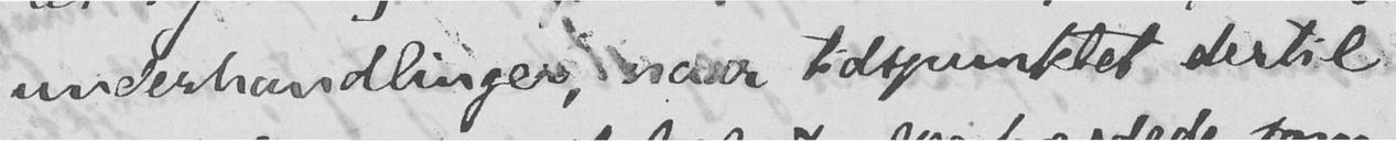underhandlinger, naar tidspunktet dertil
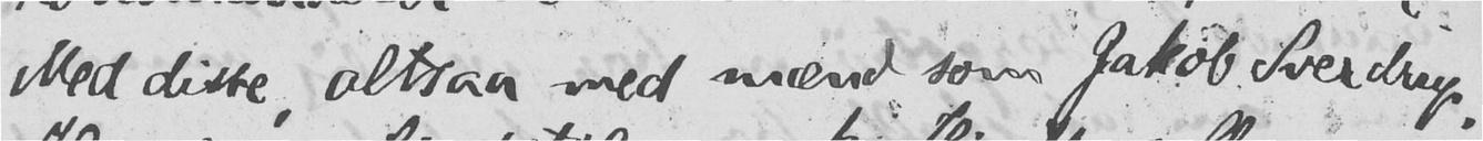Med disse, altsaa med mænd som Jakob Sverdrup,
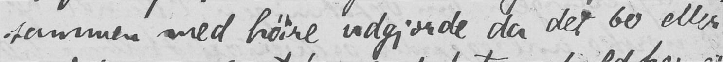sammen med høire udgjorde da det 60 eller
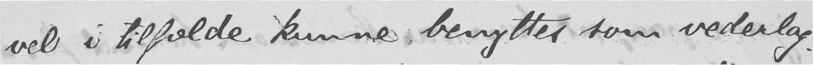vel i tilfælde kunne benyttes som vederlag.
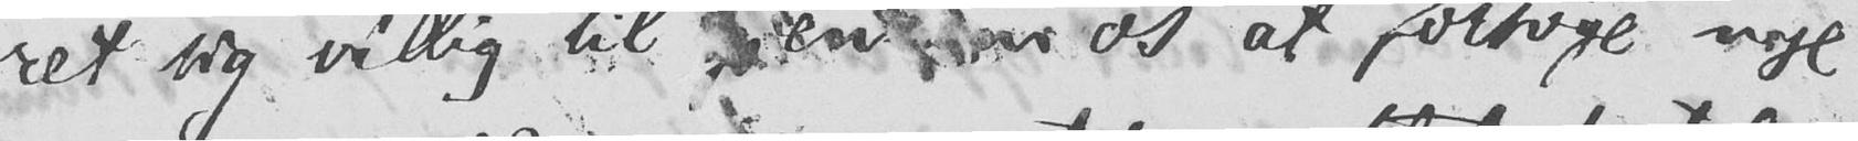ret sig villig til gjennem os at forsøge nye
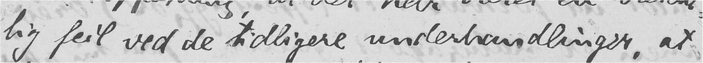lig feil ved de tidligere underhandlinger, at
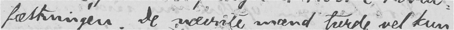fæstningen. De nævnte mænd turde vel kun-
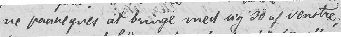ne paaregnes at bringe med sig 30 af venstre,
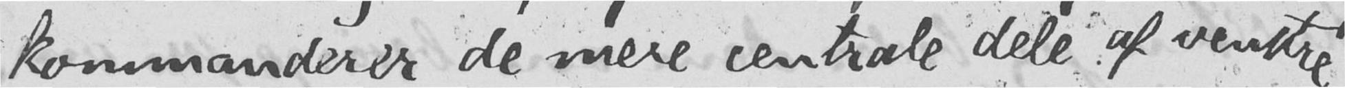kommanderer de mere centrale dele af venstre.
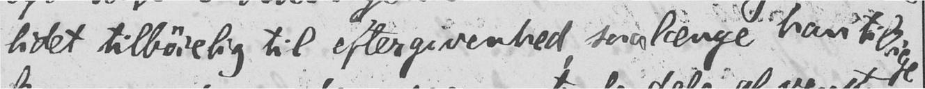lidet tilbøielig til eftergivenhed, saalænge han tillige
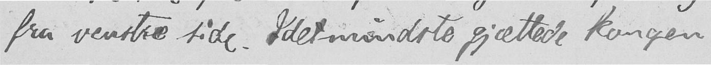fra venstre side. Idetmindste gjættede kongen
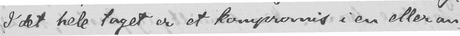I det hele taget er et kompromis i en eller an-
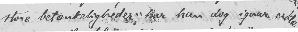store betænkeligheder, har han dog igaar erklæ-
